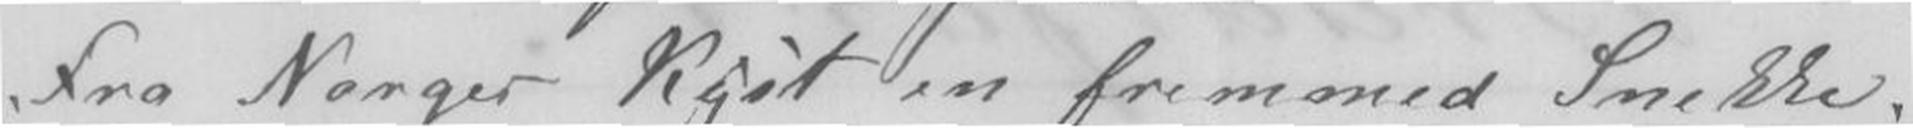fra Norges Kyst en fremaned Snekke;
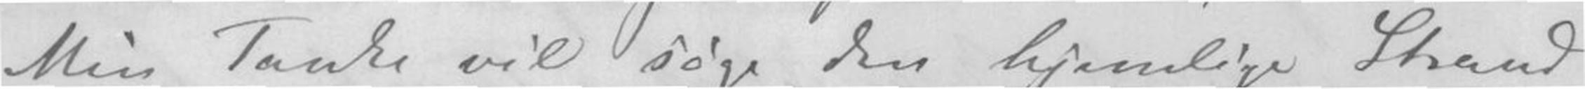Min Tanke vil søge den hjemlige Strand
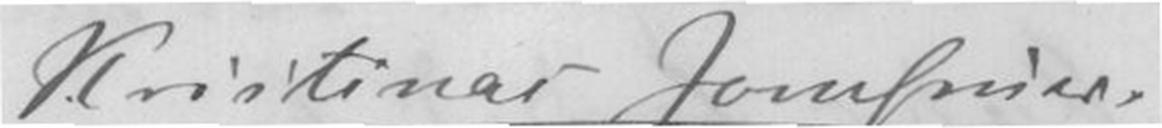Kristinas Jomfruer.
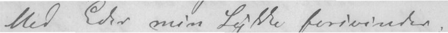Med Eder min Lykke forsvinder;
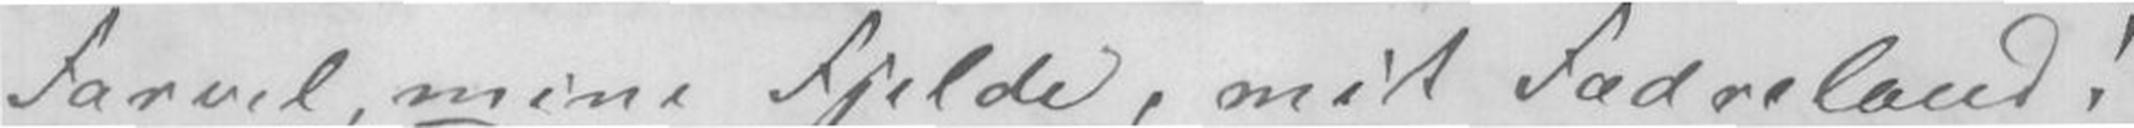Farvel, mine fjelde, mit Fædreland!
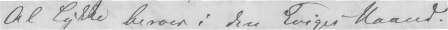Al Lykke beroer i den Eviges Haand!
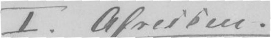I Afreisen.
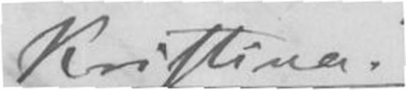Kristina.
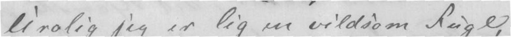Ei rolig jeg er lig en vildsom Fugl,
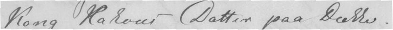Kong Hakons - Datter paa Dække.
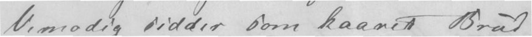Vemodig sidder som kaaret Brud
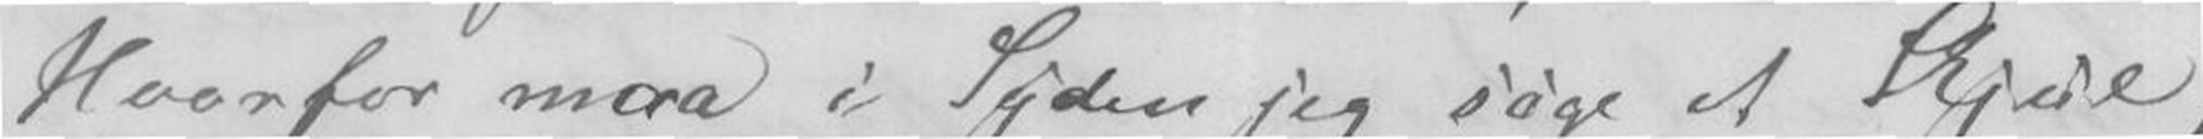Hvorfor maa i Syden jeg søge et Skjul,
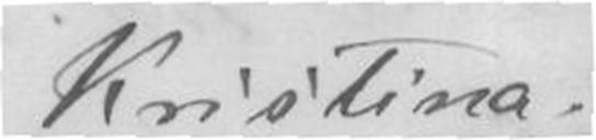Kristina.
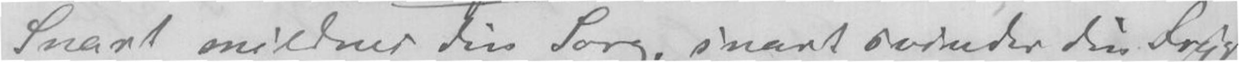Snart mildner din Sorg, snart svinder din frygt;
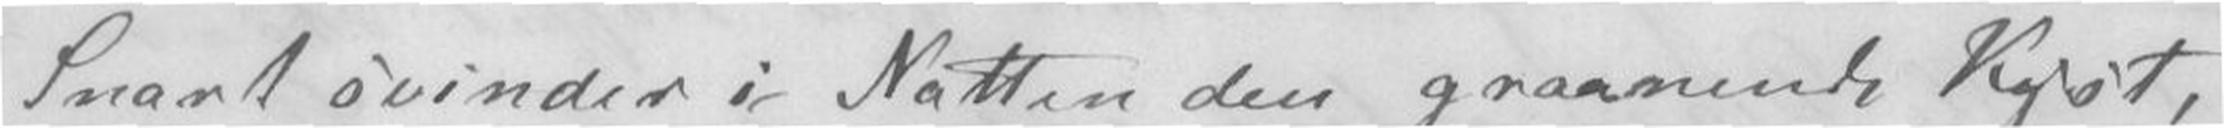Snart svinder i, natten den graanende Kyst,
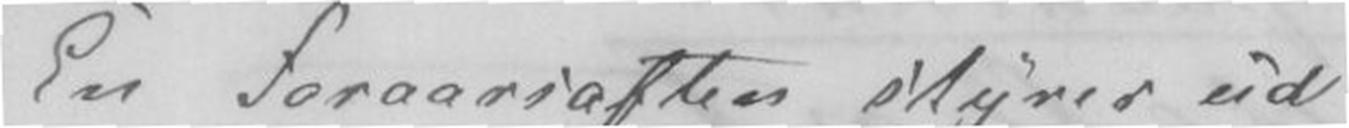En foraarsaften styres ud
